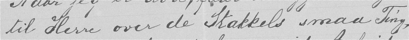til Herre over de Stakkels smaa Ting,
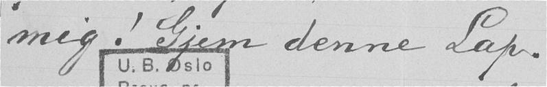mig! Gjem denne Lap.
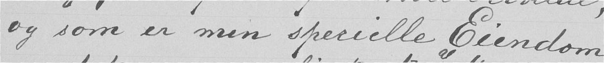og som er min specielle Eiendom
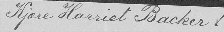Kjære Harriet Backer!
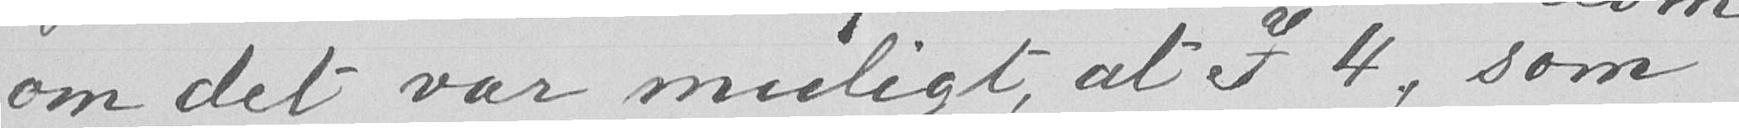om det var muligt, at I 4, som
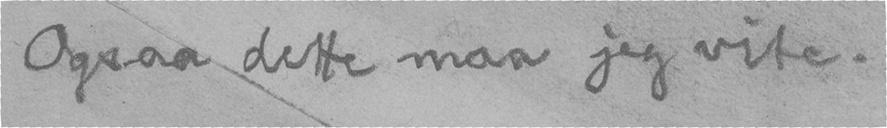Ogsaa dette maa jeg vite.
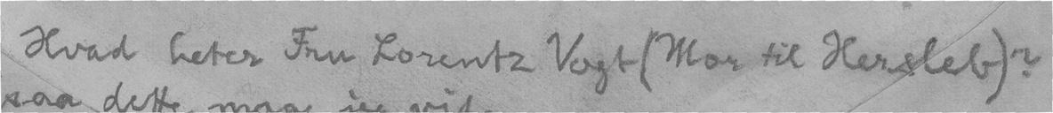Hvad heter Fru Lorentz Vogt (Mor til Hersleb)?
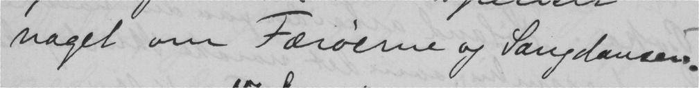noget om Færøerne og Sangdanser.
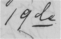19de
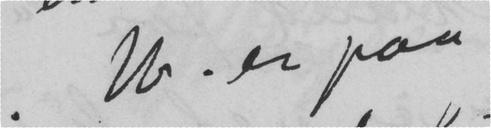. W. er paa
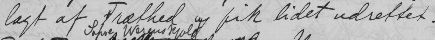lagt af Træthed og fik lidet udrettet.
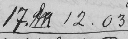17. 12.03
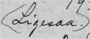(Ligesaa.)
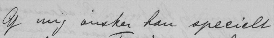af mig ønsker han specielt
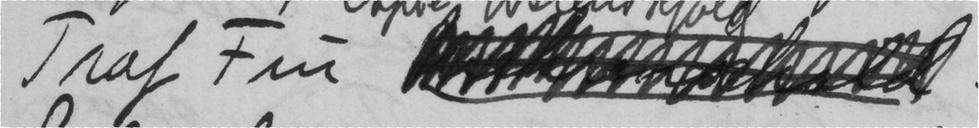Traf Fru
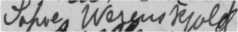Sofie Werenskjold
